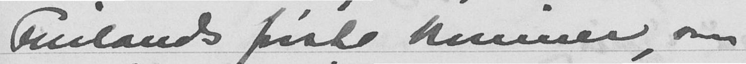Finlands friske kvinner, som
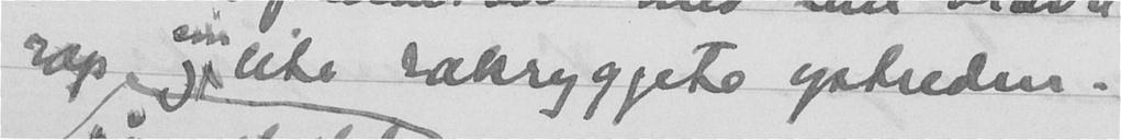rop, og lite rakryggete optreden.
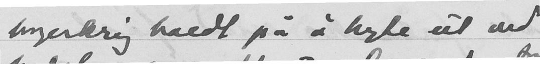borgerkrig holdt på å bryte ut ved
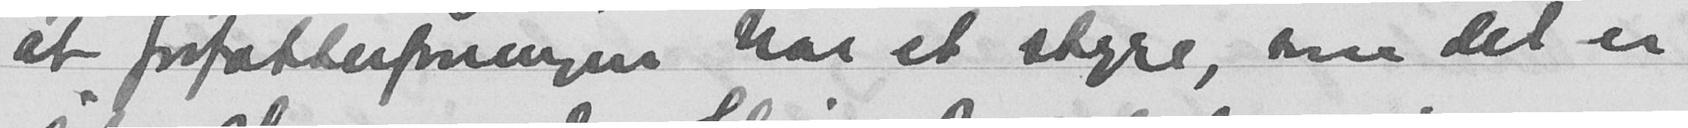at forfatterforeningen har et styre, som det er
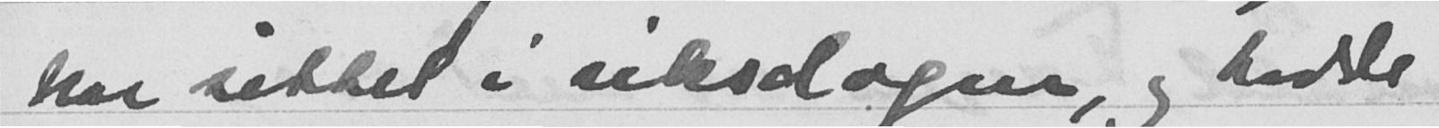har sittet i redaksjonen, og hadde
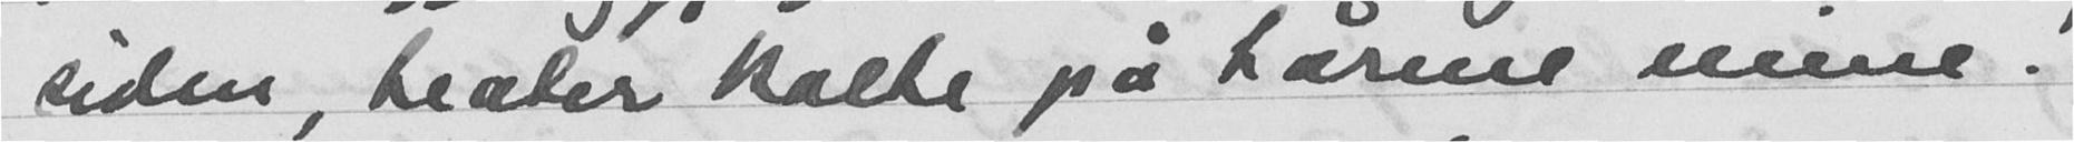siden, teater kalte på tårene mine.
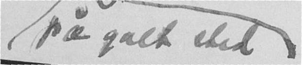på galt sted
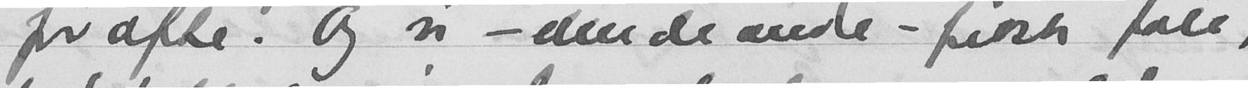for ofte. Og vi - eller de andre - fikk tåle,
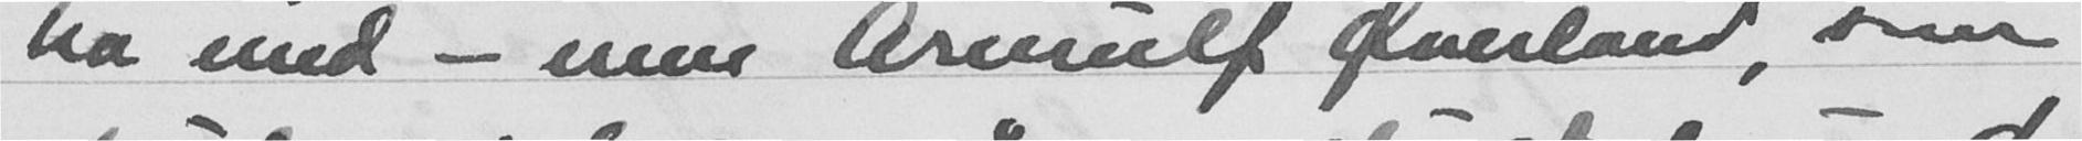ha med - men Arnult Øverland, som
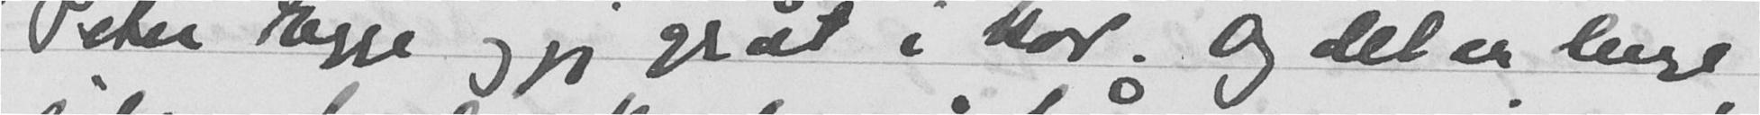Peter Egge og jeg gråt i kor. Og det er lenge
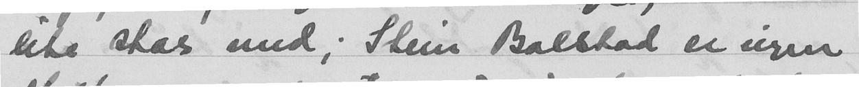lite stas med; Stein Balstad er igjen
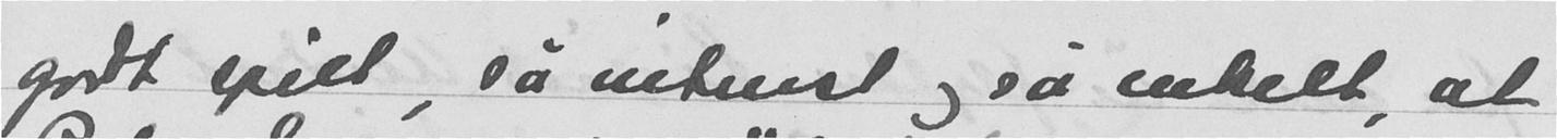godt spilt, så intenst og så enkelt, at
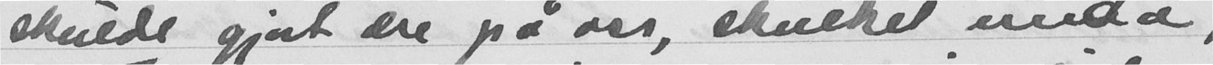skulde gjort ære på oss, skulket unda,
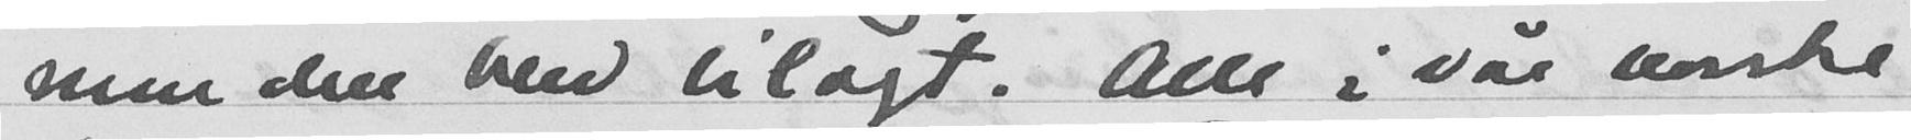men den blev tilagt. Alle i vår norske
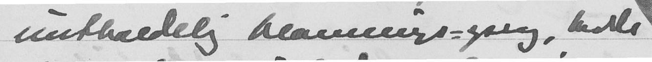uutholdelig blannngs-gsng, havde
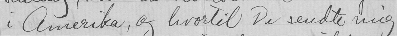i Amerika, og hvortil De sendte mig
